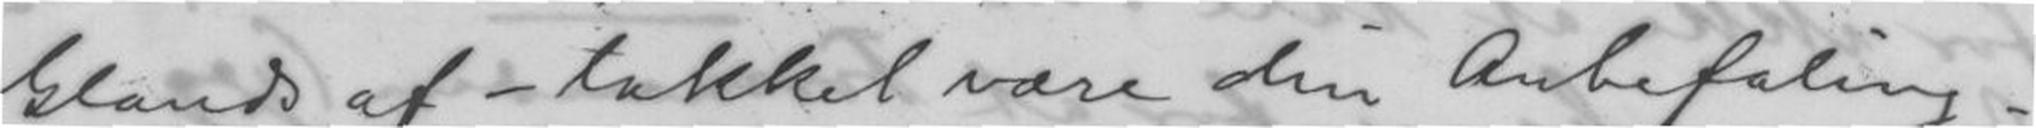Glands af - takket være din Anbefaling -
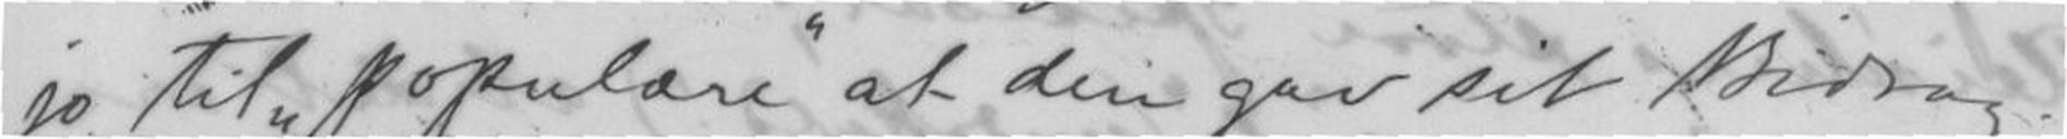jo til populære at den gav sit Bidrag.
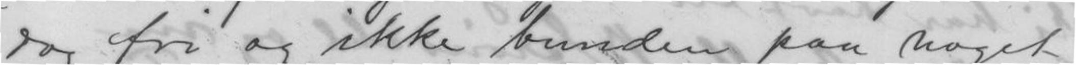dog fri og ikke bunden paa noget
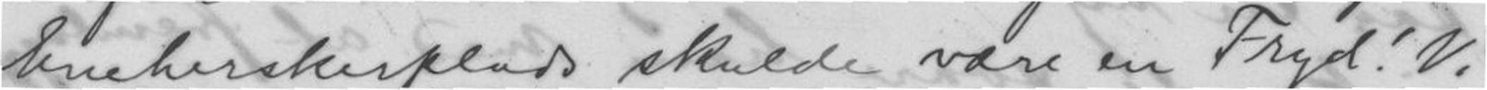Eneherskerplads skulde være en Fryd! Vi
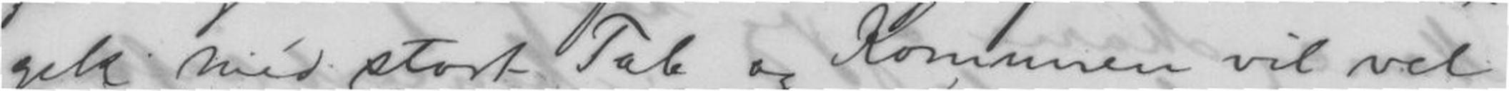gik med stort Tab og Komunen vil vel
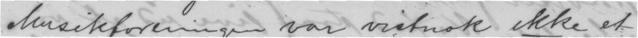Musikforeningen var vistnok ikke et
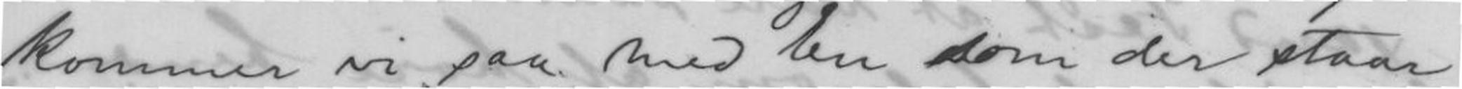kommer vi saa med En som der staar
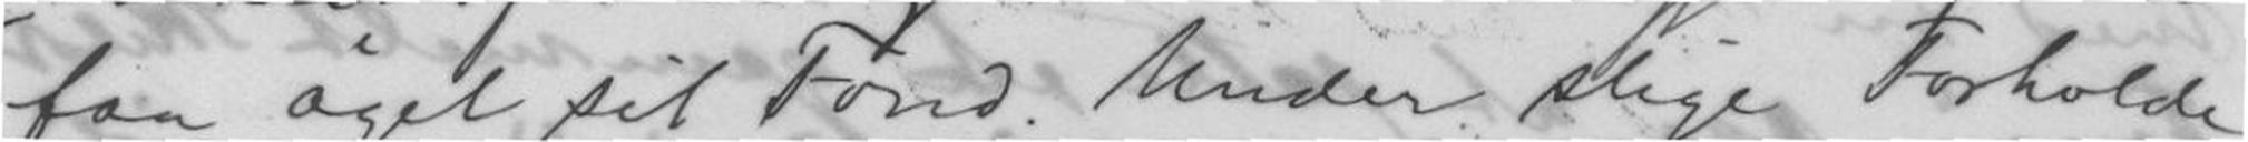faa øget sit Fond. Under slige Forholde
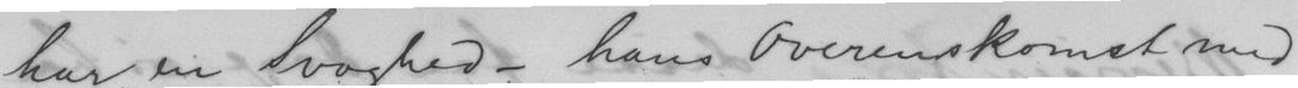har en Svaghed - hans Overenskomst med
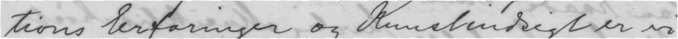tions Erfaringer og Kunstindsigt er vi
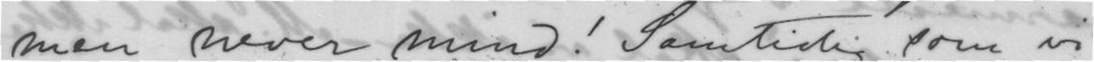men never mind! Samtidig som vi
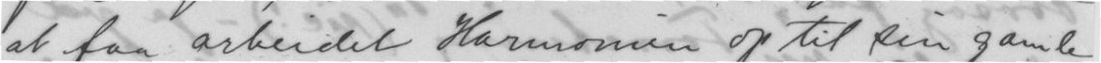at faa arbeidet Harmonien op til sin gamle
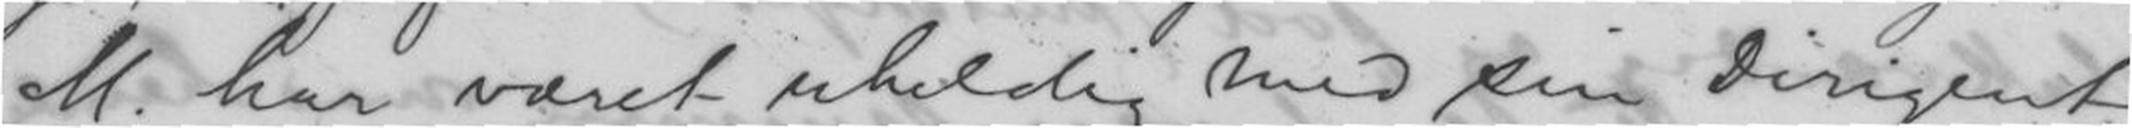M. har været uheldig med sin Dirigent;
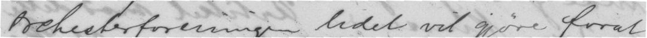Orchesterforeningen lidet vil gjøre forat
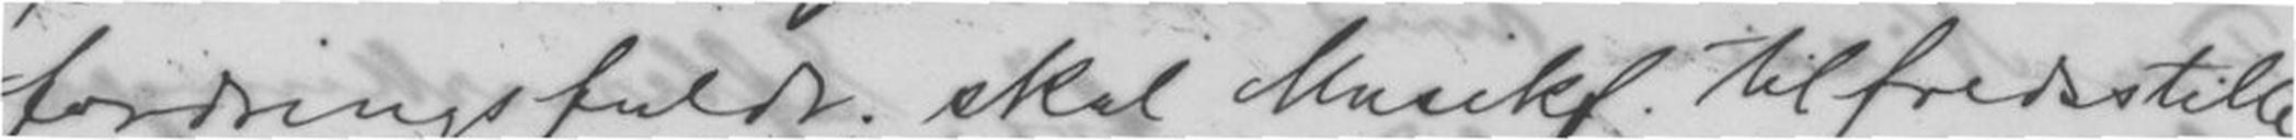fordringsfuldt; skal Musikf. tilfredsstille
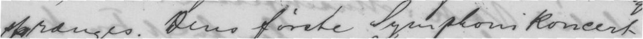sprænges. Deres første Symphonikoncert
